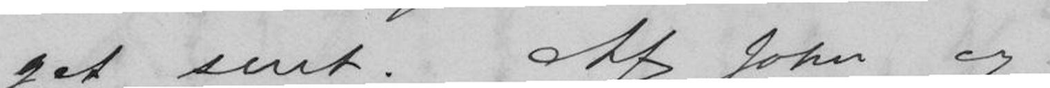get sent. Af John og
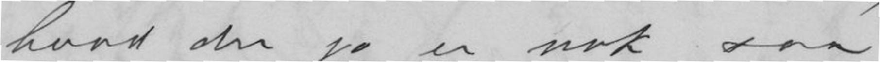hvad der jo er nok saa
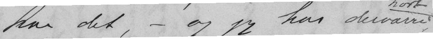har det, - og jeg har desværre
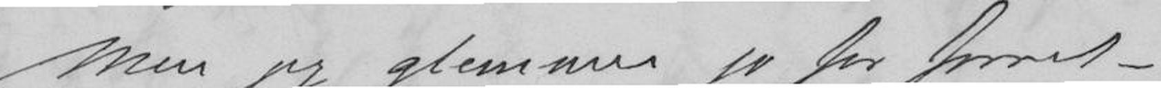Men jeg glemmer jo for forret-
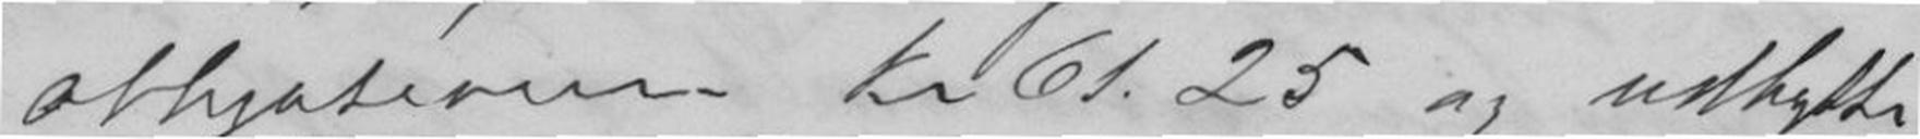obligatione kr 61.25 og udbytte
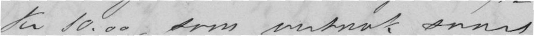kr 10.00, som vistnok snart
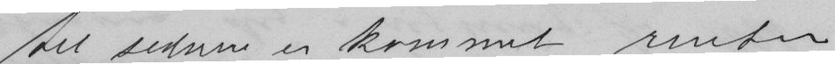til siderne er kommet renter
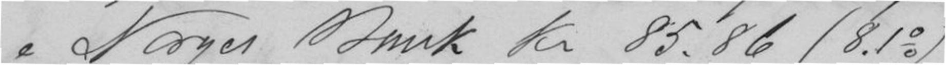i Norges Bank kr 85.86 (8.1%).
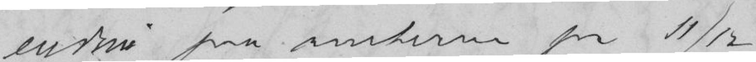endnu paa renterne pr 11/12
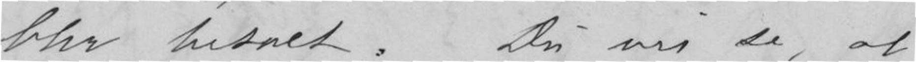blir betalt. Du vil se, at
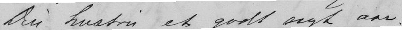Din hustru et godt nyt aar.
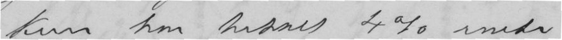kun har betalt 4% rente,
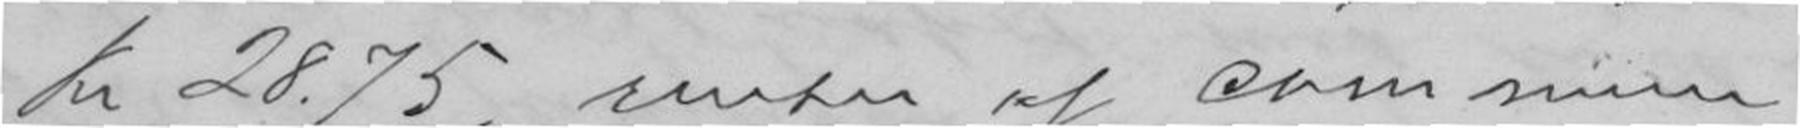kr 28.75, renter af commune-
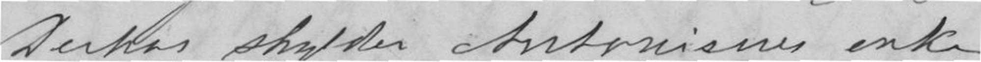Derhos skylder Antonisens enke
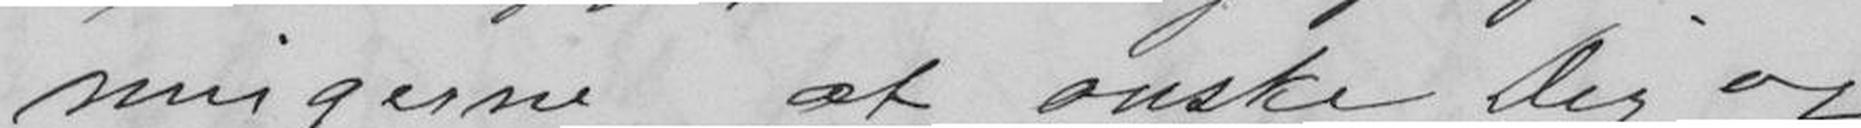ningerne at ønske Dig og
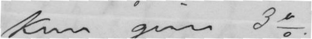kan give 3%.
